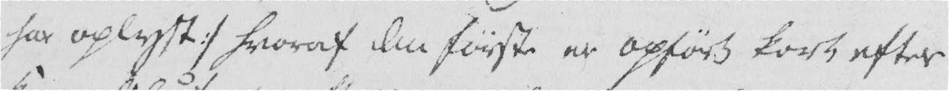har oplyst :/ hvoraf deen første er opført kort efter
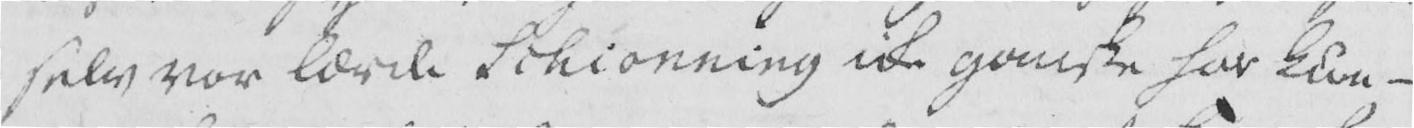selv vor lærde Schiønning ikke ganske har kun-
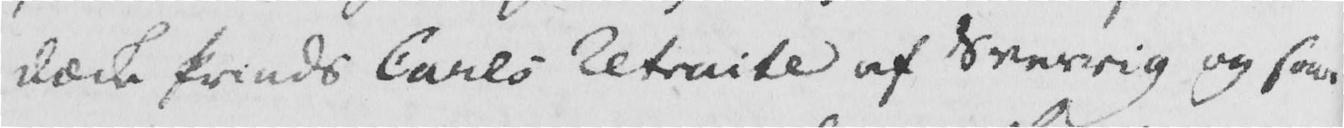dæcke Prinds Carls Retraite af Sverrig og saa
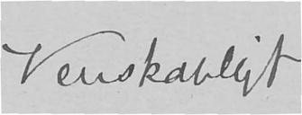Venskabeligt
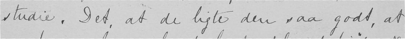studie. Det at de ligte den saa godt, at
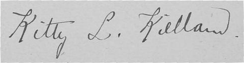Kitty L. Kielland.
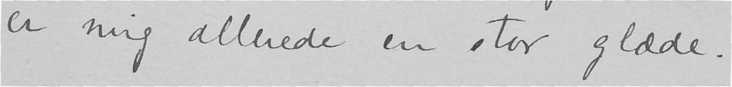er mig allerede en stor glæde.
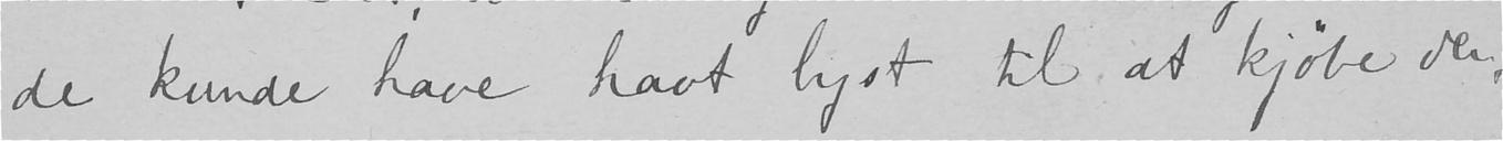de kunde have havt lyst til at kjøbe den,
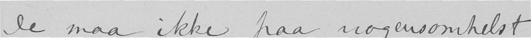De maa ikke paa nogensomhelst
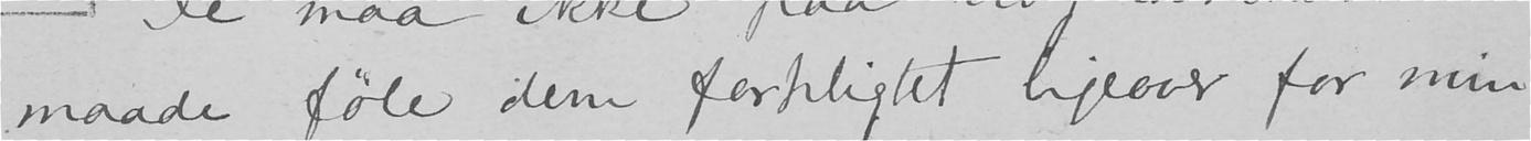maade føle dem forpligtet ligeover for min
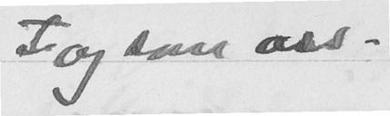Iag som oss-
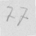77
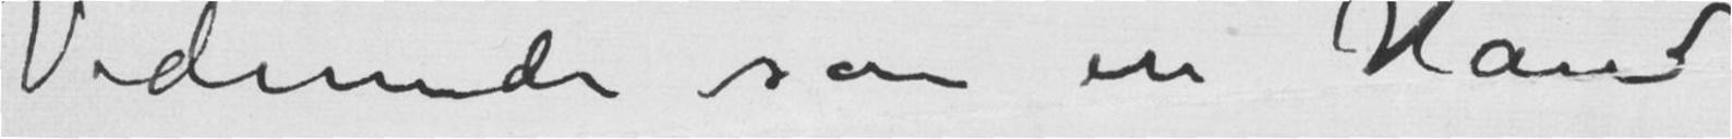Vidunder som en Hånd
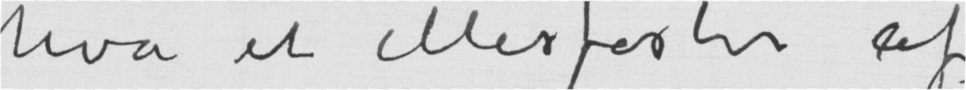hva et Misfoster af
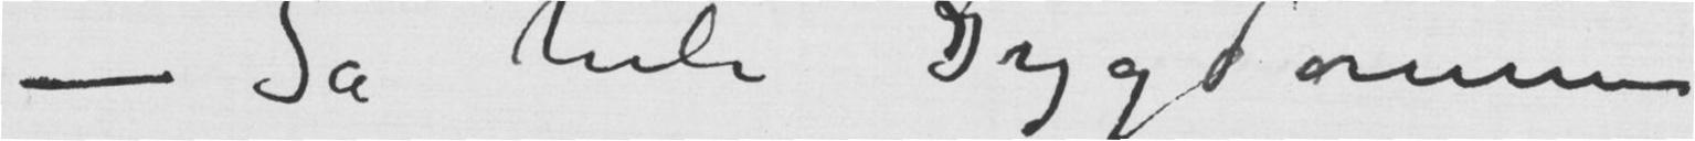– Så hele Sygdommen
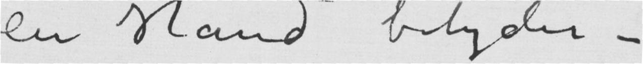en Hånd betyder –
